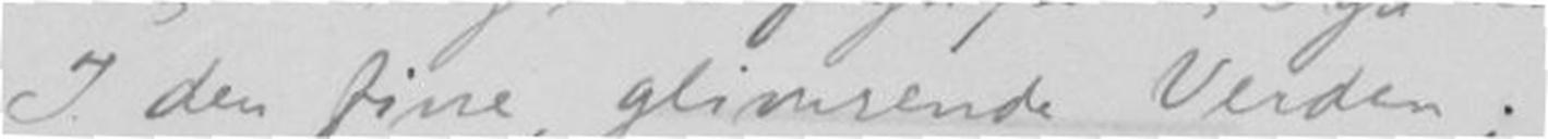I den fine, glinsende Verden;
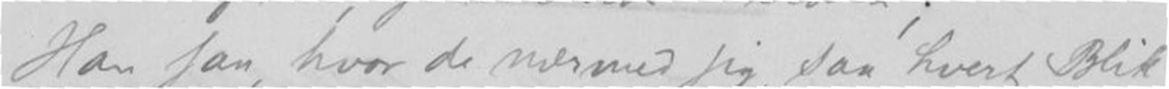Han saa, hvor de nærmed sig, saa hvert Blik,
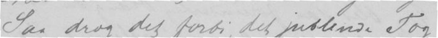Saa drog det forbi, det jublende Tog
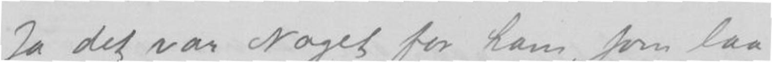Ja det var Noget for ham, som laa
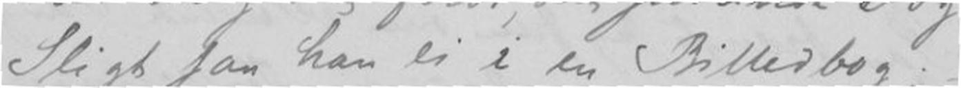Sligt saa han ei i en Billedbog; -
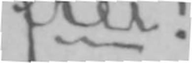frei!
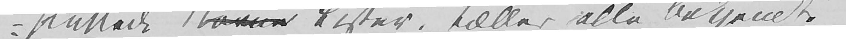sluttede Navne Lister, tæller alle bekjendte
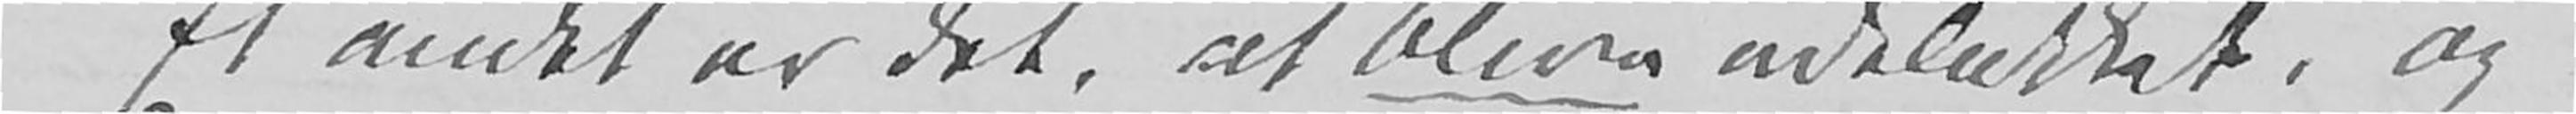Et andet er det, at blive udelukket, og
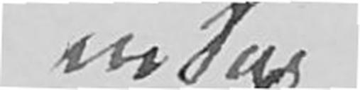en Sag
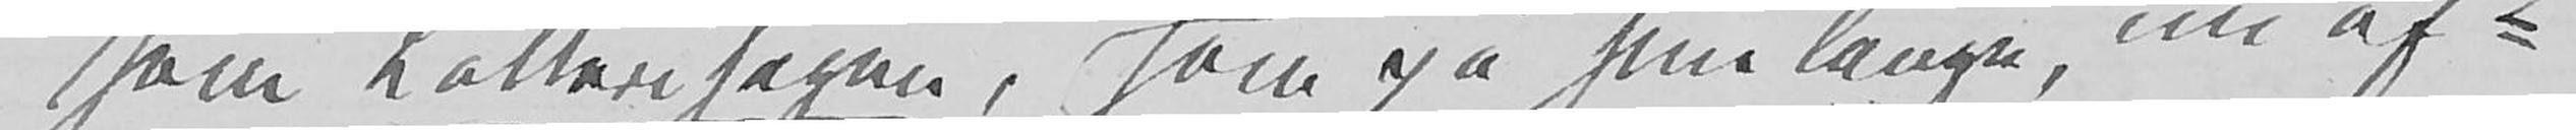som Lotterisagen, som på sine lange, nu af¬
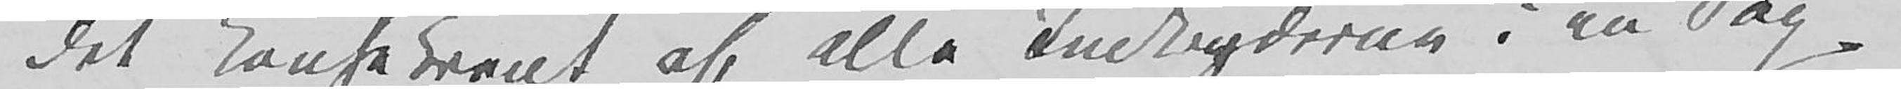det konsekvent af alle Indbyderne i en Sag –
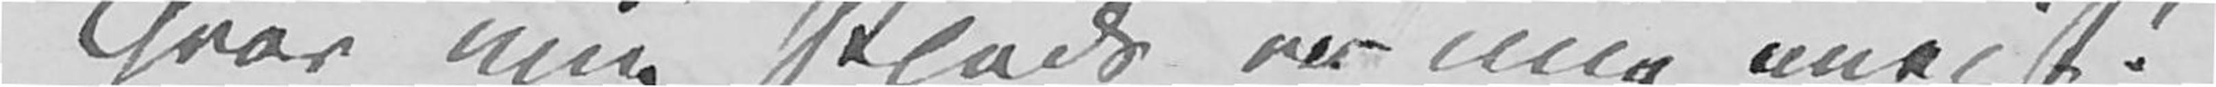hvor min Plads er mig anvist!
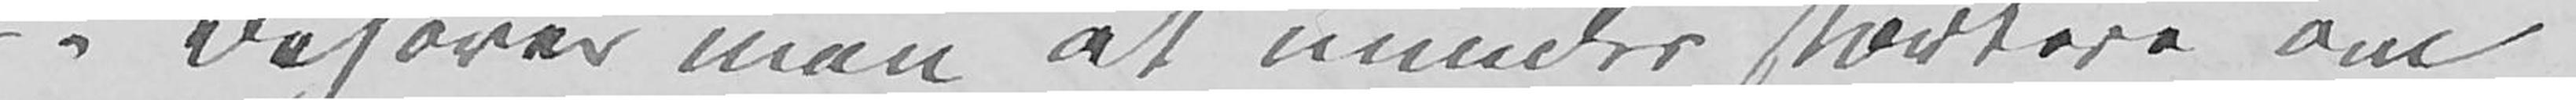–, behøver man at mindes stærkere om
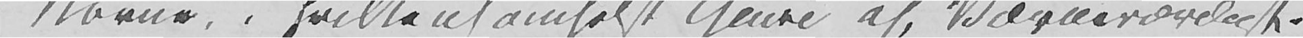Navne, i hvilkensomhelst Genre af Nævneværdigt
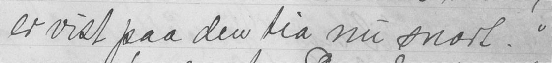er vist paa den tia nu snart. "
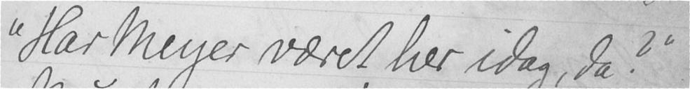"Har Meyer været her idag, da?"
